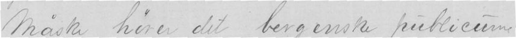Måske hører det bergenske publicum
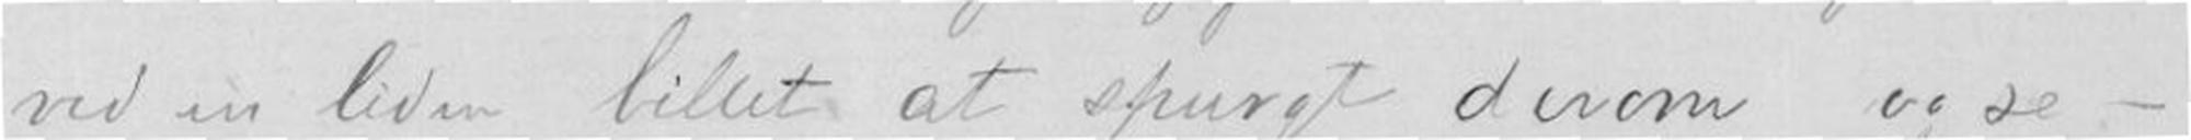ved en liden billet at spurgt derom og se -
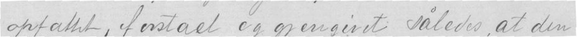opfattet, forstået og gjengivet således, at den
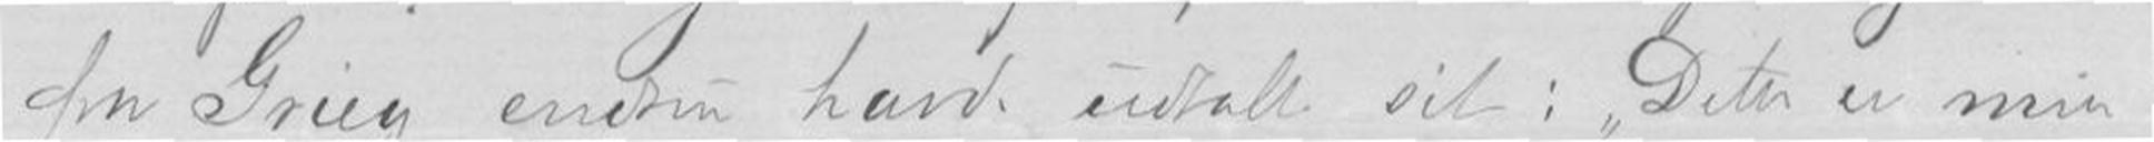fru Grieg endnu havd udtalt sit: Dette er min
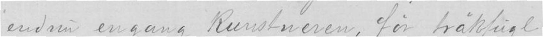endnu engang kunstneren, før träkfugl
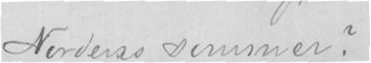Nordens sommer? -
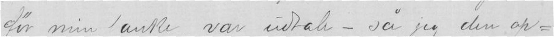før min tanke var udtale - så jeg den op-
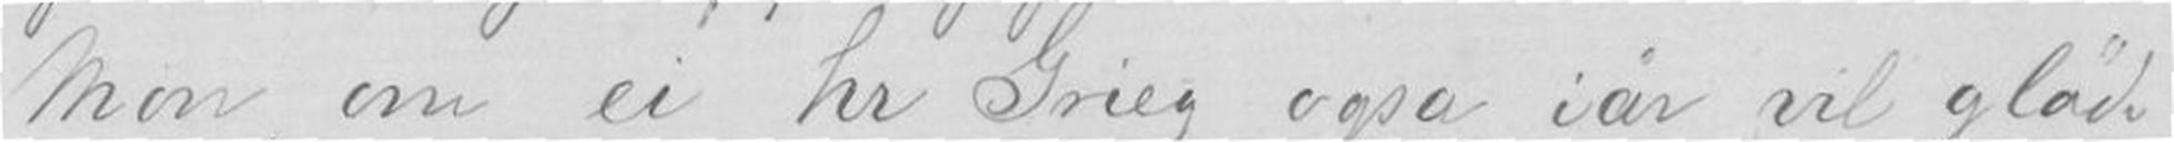Mon, om ei hr Grieg også iår vil gläde
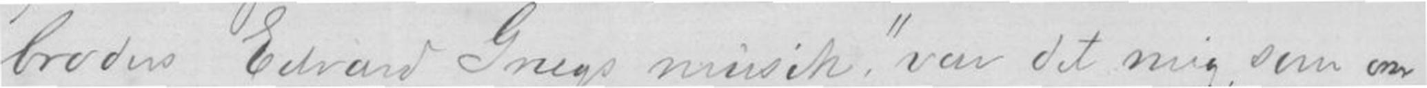broders Edvard Grieg musik, var det mig som om
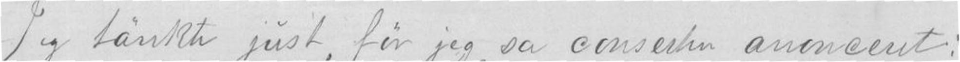Jeg tänkte just, før jeg så conserten anonceret:
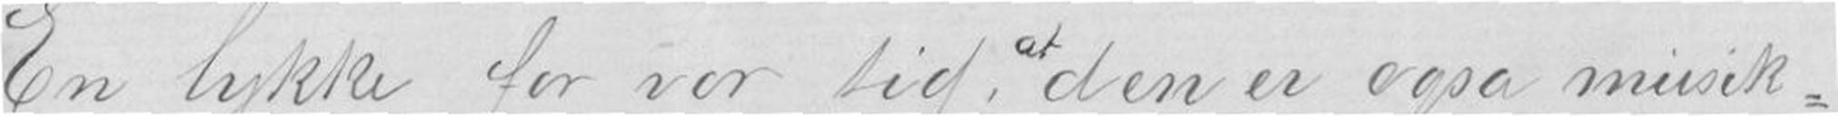En lykke for vor tid, at den er ogsa musik}
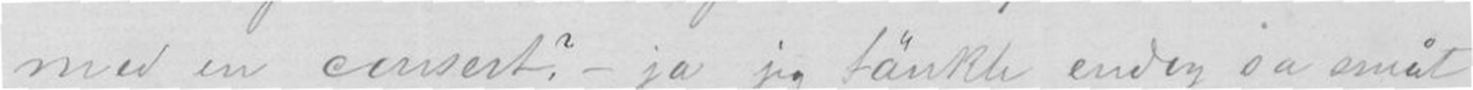med en consert? - ja jeg tänkte endog så småt
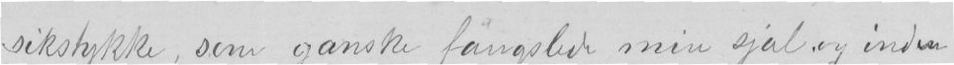sikstykke, som ganske fängslede min sjæl og inden
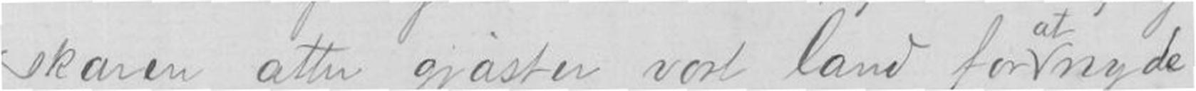skaren atter gjæster vort land for nyde
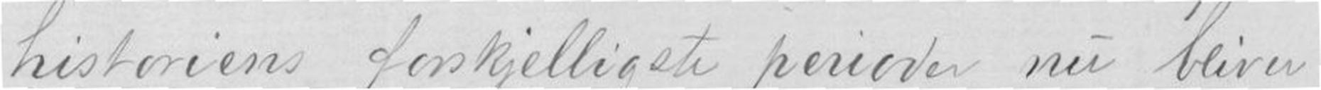historiens forskjelligste perioder nu bliver
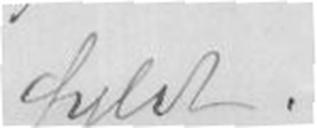fyldt.
